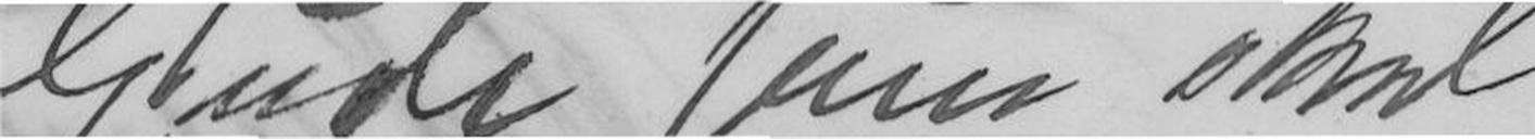Gude som skal
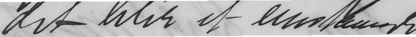det blir et enestaaende
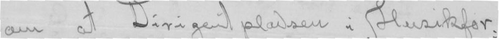om at Dirigentpladsen i Musikfor-
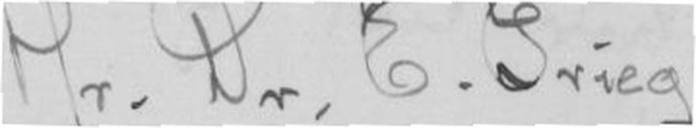Hr. Dr. E. Grieg!
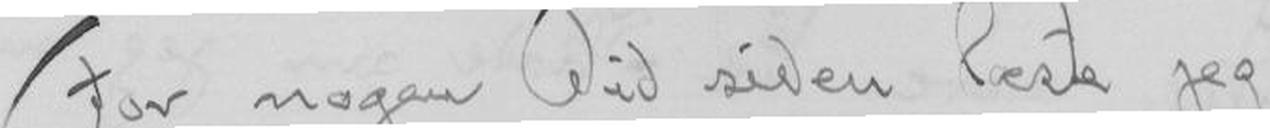For nogen Tid siden leste jeg
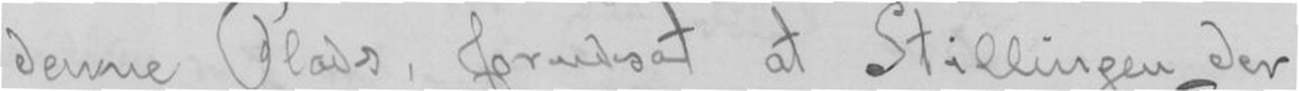denne Plads, forudsat at Stillingen der
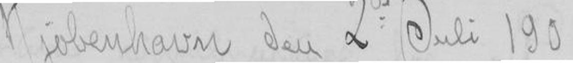Kjøbenhavn den 2de Juli 1901
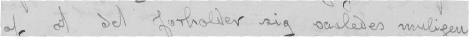af at det forholder sig saaledes muligen
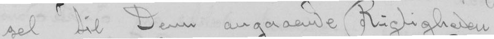sel til Dem angaaende Rigtigheden
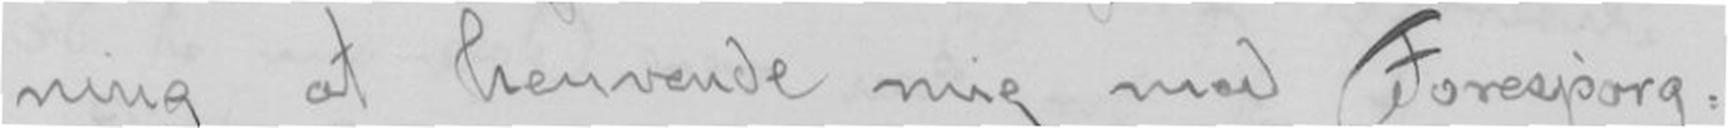ning at henvende mig med Forespørg-
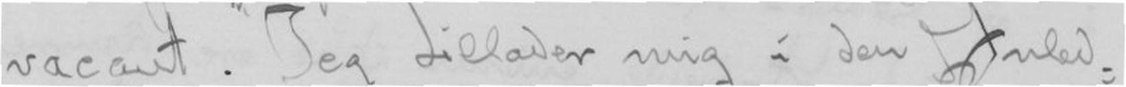vacant. Jeg tillader mig i den Anled-
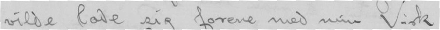vilde lade sig forene med min Virk-
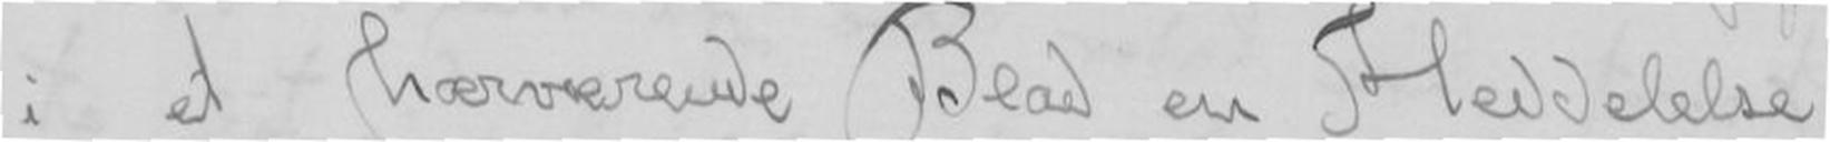i et hærværende Blad en Meddelelse
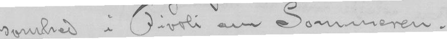somhed i Tivoli over Sommeren.
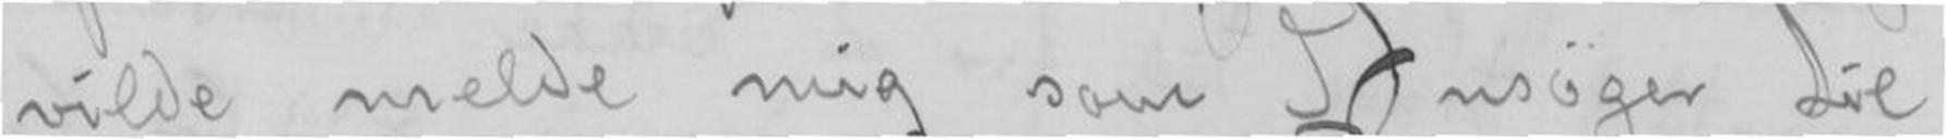vilde melde mig som Ansøger til
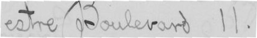Vestre Boulevard 11.
